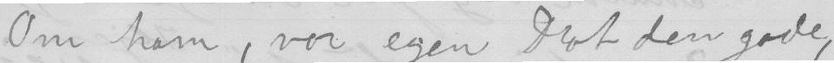Om ham, vor egen Drot den gode
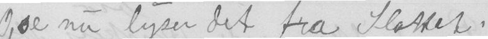O, se nu lyser det fra Slottet.
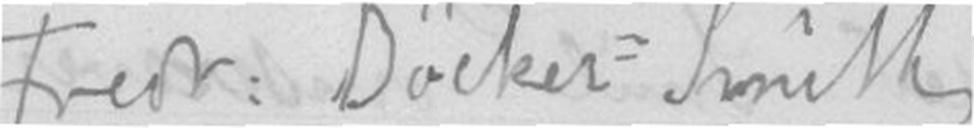Fredr. Döcker=Smith
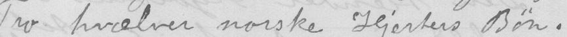Tro hvælver norske Hjerters Bøn.
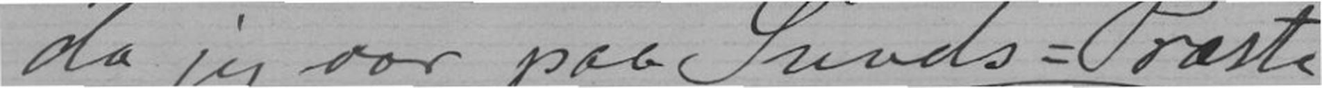da jeg var paa Sunds-Præste-
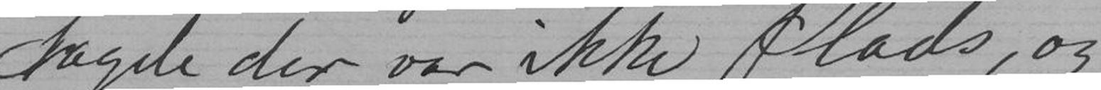Sagde der var ikke Plads, og
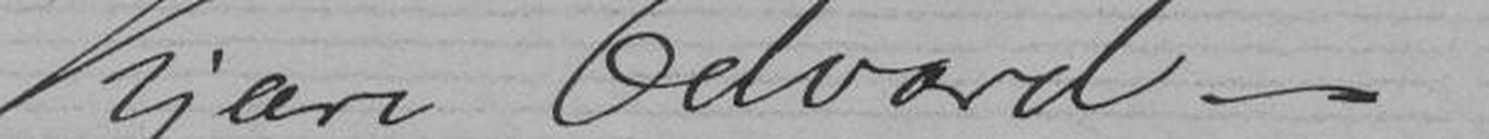Kjære Edvard.
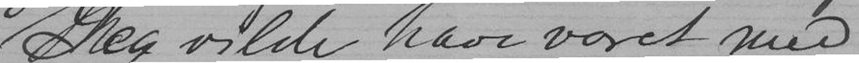Jeg vilde have været med
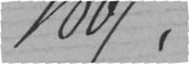1867.
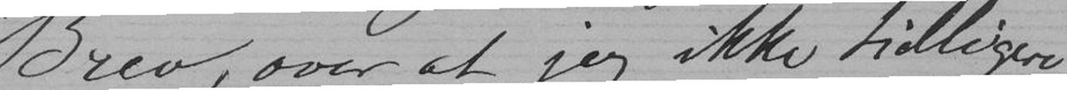Brev, over at jeg ikke tidligere
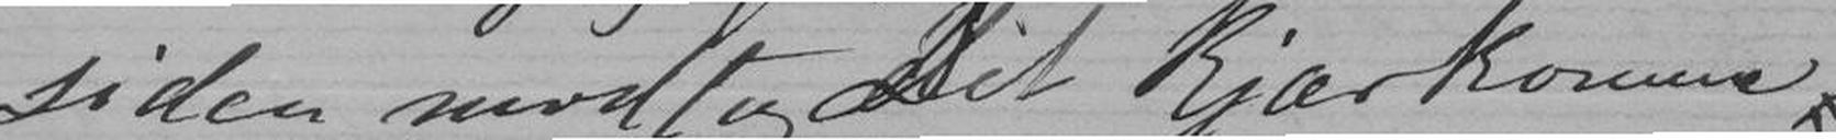siden modtog Dit kjærkomne
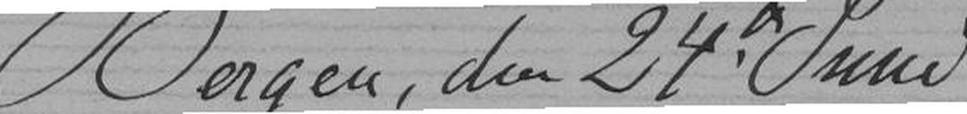Bergen, den 24de Juni
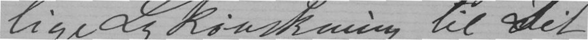lige Lykønskning til Dit
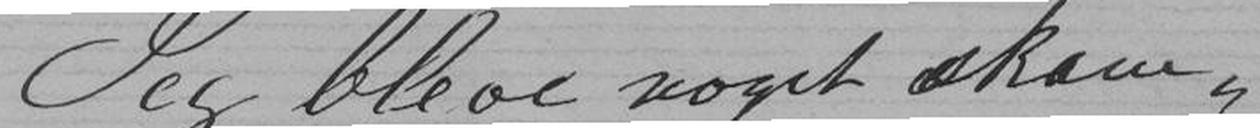Jeg bleve noget skam-
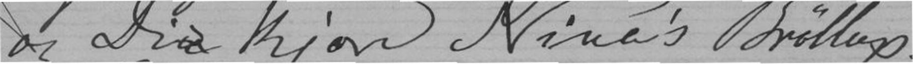og Din kjære Nina's Brøllup.
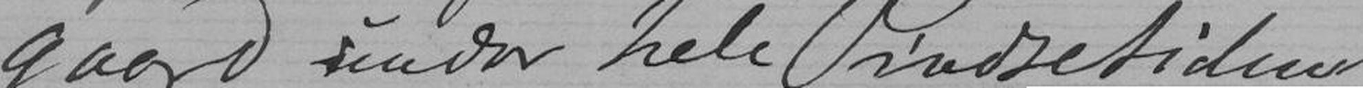gaard under hele Pindstetiden
